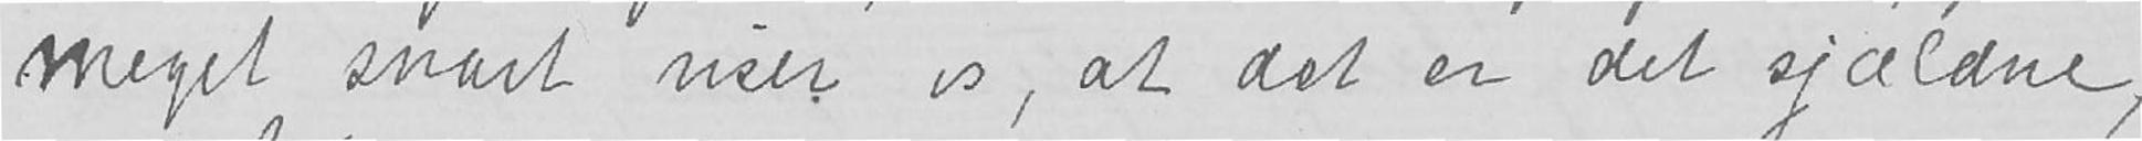meget snart viser os, at det er det sjældne,
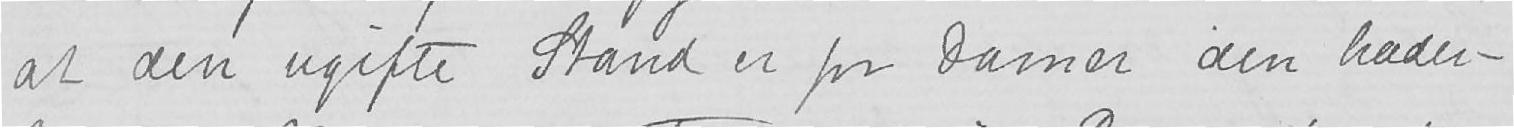at den ugifte Stand er for Damer den hæder-
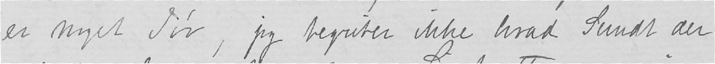er noget Tøv, jeg begriber ikke hvad Sundt der
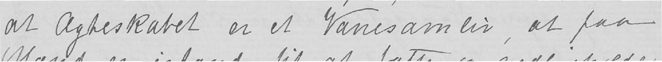at Ægteskabet er et Vanesamliv, at faa
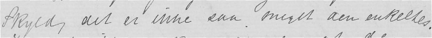Skyld, det er ikke saa meget den enkeltes.
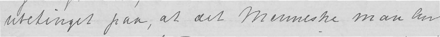ubetinget paa, at det Menneske man har
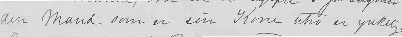den Mand som er sin Kone utro er ynkelig.
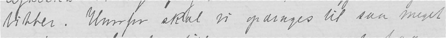bitter. Hvorfor skal vi opdrages til saa meget
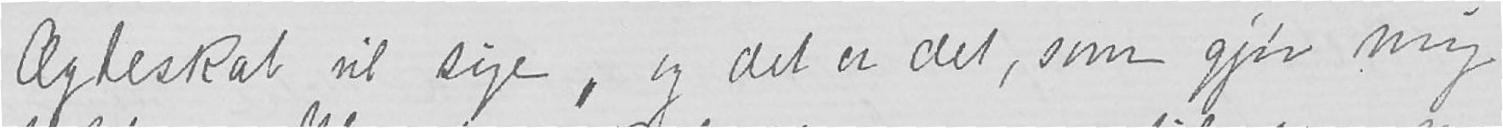Ægteskab vil sige, og det er det, som gjør mig
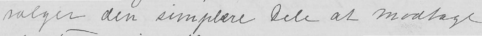vælger den simplere Dele at modtage
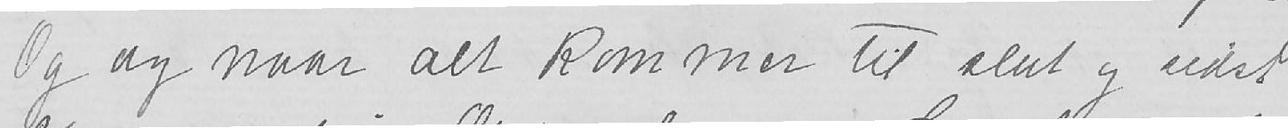Og dog naar alt kommer til slut og sidst
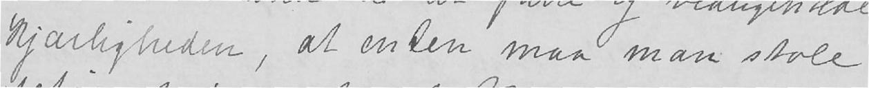Kjærligheden, at enten maa man stole
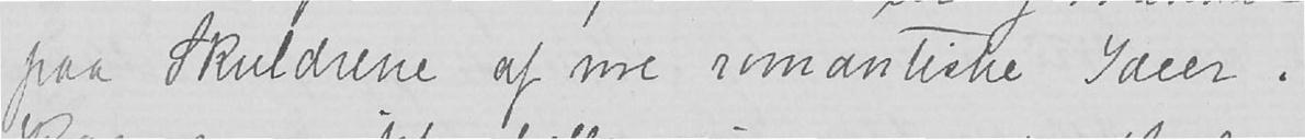paa Skuldrene af vore romantiske Ideer.
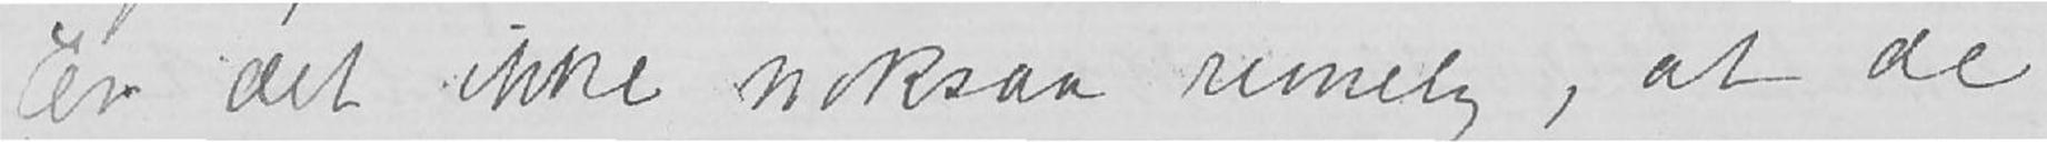Er det ikke noksaa rimelig, at de
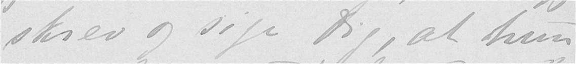skrev og sige dig, at hun
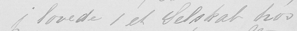jeg lovede i et Selskab hos
